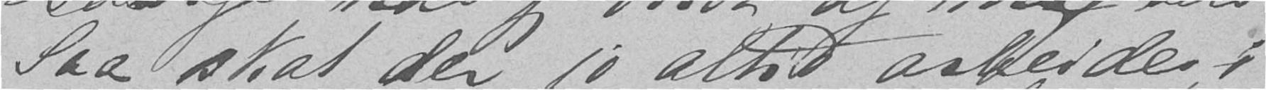Saa skal der jo altid arbeides i
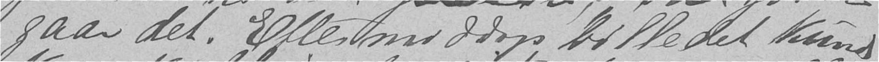gaar det. Ettermiddagsbilledet kunde
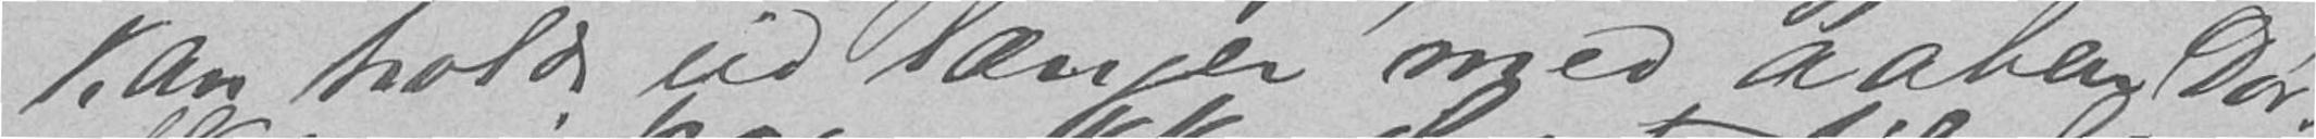kan holde ud længer med aaben Dør.
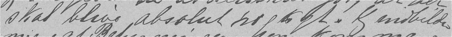skal blive absolut rigtigt. Jeg indbilder
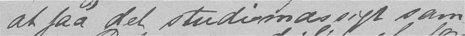at faa det studiemæssigt sam
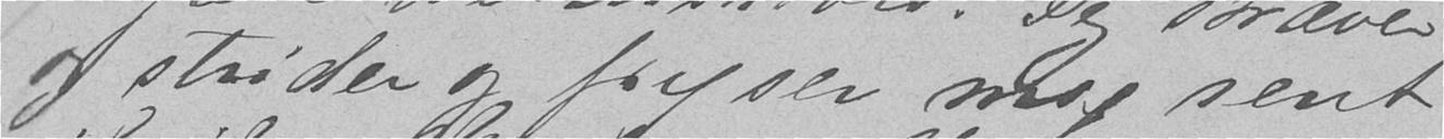og strider og fryser mig rent
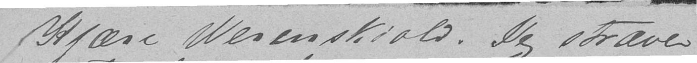Kjære Werenskiold. Jeg stræver
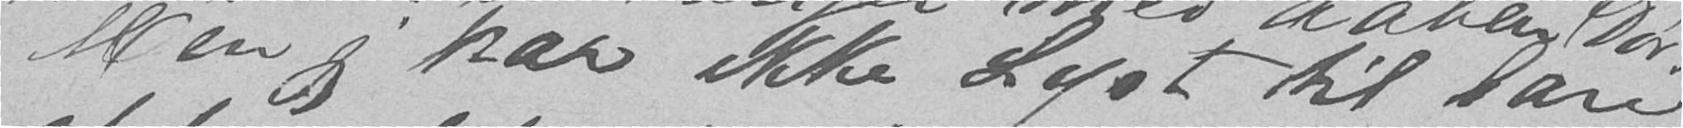Men jeg har ikke Lyst til bare
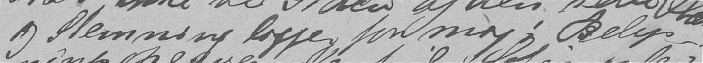og Stemning ligger for mig i Belys-
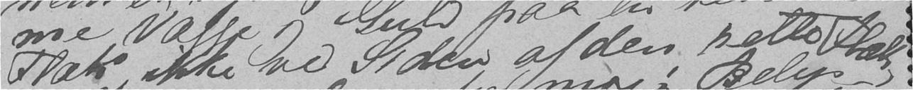Flæk, ikke ved Siden af den rette Flæk
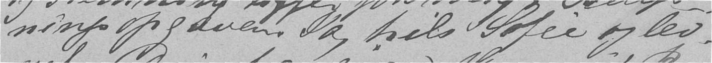ninsopgaven. Ja, hils Sofie og lev
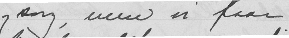og sorg, men vi faar
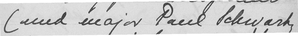(med major Paul Schwartz
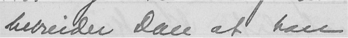bebreider Don at han
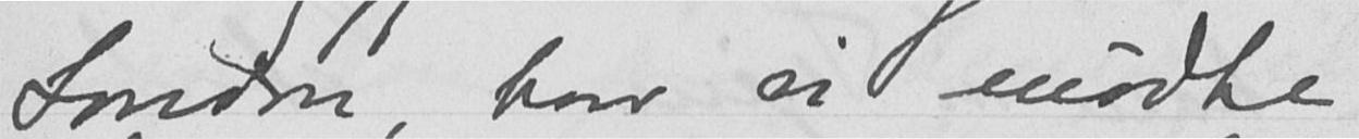London, hvor vi mødte
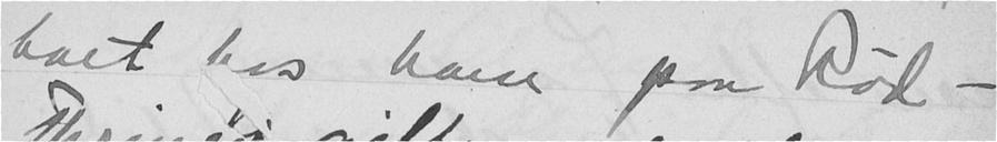boet hos ham paa Rød -
